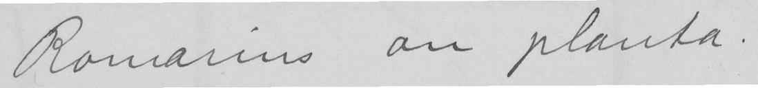Romarins an planta.
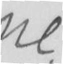ne
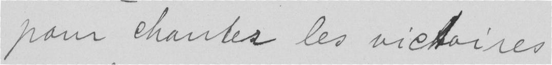pom chanter les victoires
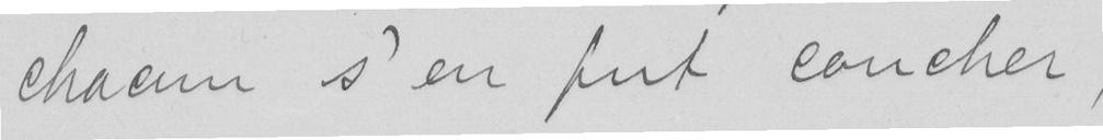Chaunm s'en fut Concher,
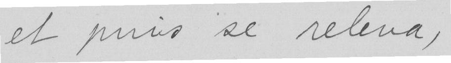et prius se releva,
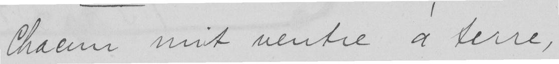Chacim mit ventre á terre,
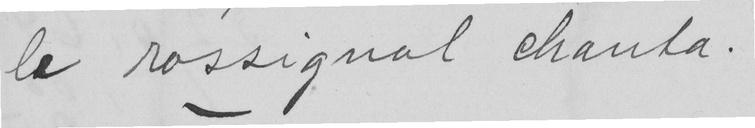le rossignal Chanta.
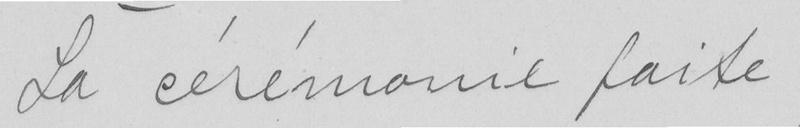La cérémonie faite
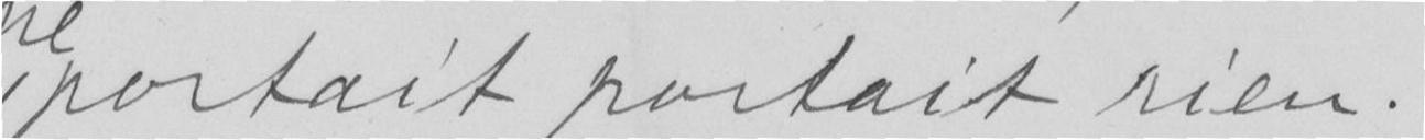portait portait rien.
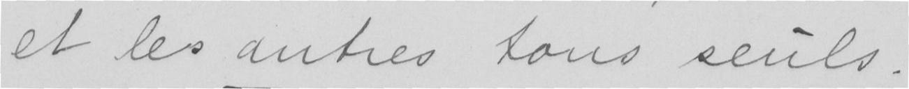et les antres tons seuls.
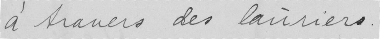á travers des lauriers.
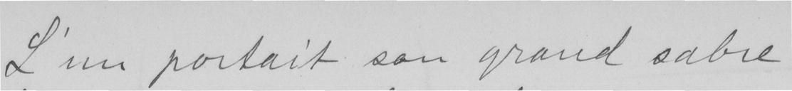L'un portait son grand sabre
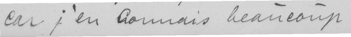Car j'en cormais beancoup
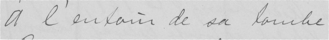Á l'entain de sa tombe
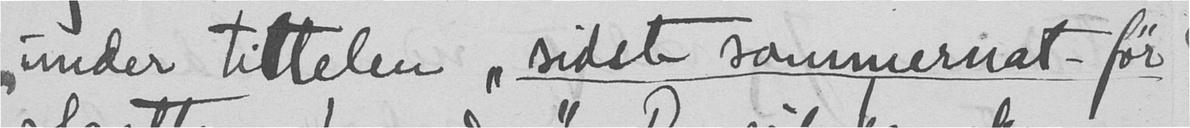under tittelen "sidst sommernat - før
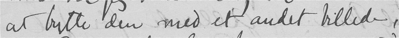at bytte den med et andet billed,
